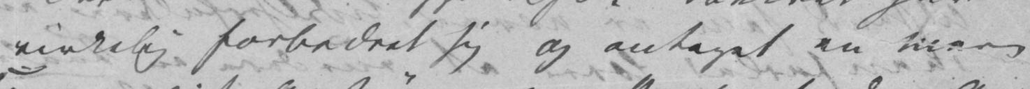virkelig forbedret sig og antaget en mere
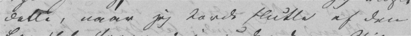dette, naar jeg torde slutte af den
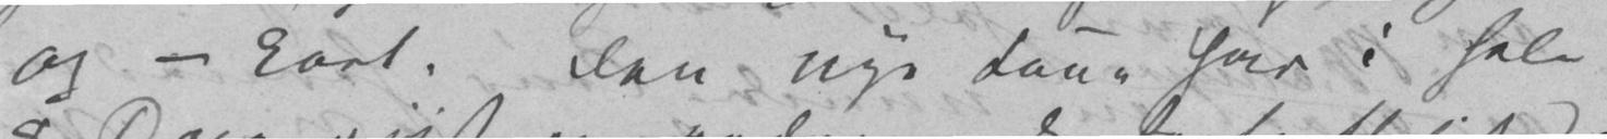og – kort. Den nye Kone har i hele
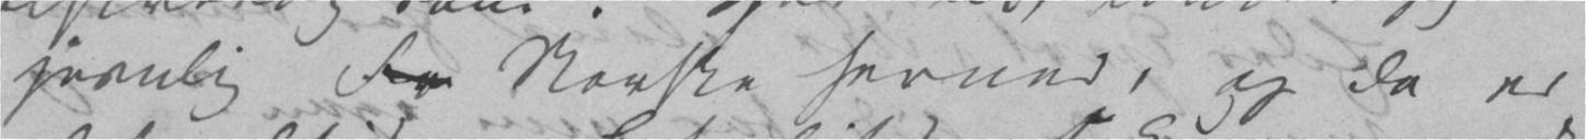jevnlig Fr Norske herned, og da er
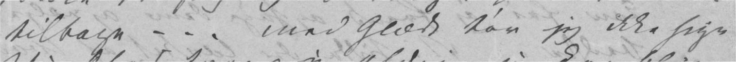tilbage – – – med Glæde tør jeg ikke sige
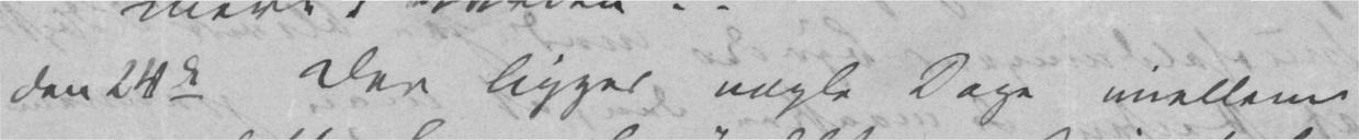Den 24de Der ligger nogle Dage imellem
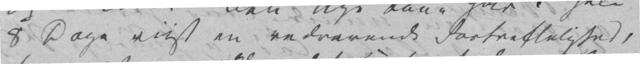8 Dage viist en vedvarende Fortreffelighed,
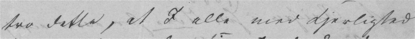tro dette, at I alle med Kjerlighed
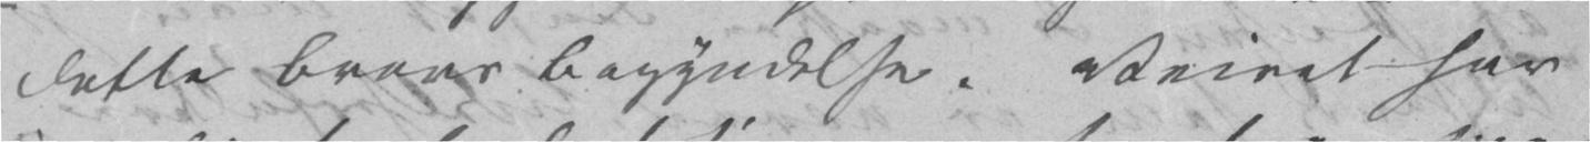dette Brevs Begyndelse. Veiret har
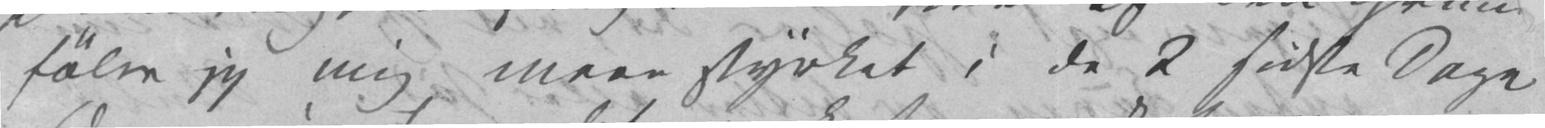føler jeg mig meer styrket i de 2 sidste Dage
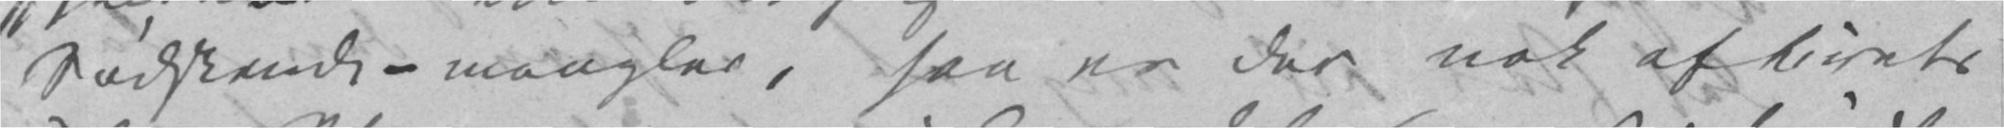Sødskende – mangler, saa er der nok af Livets
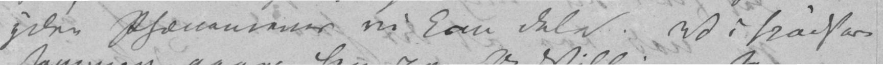ydre Phænomener vi kan dele. Vi spadsere
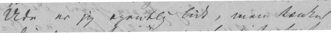Ude er jeg egentlig lidt, men tænker
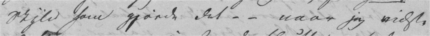Skyld som gjorde det – – naar jeg vidste
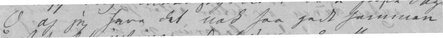O og jeg have det nok saa godt sammen
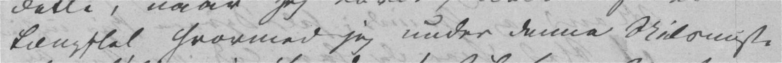Længsel hvormed jeg under denne Skilsmisse
